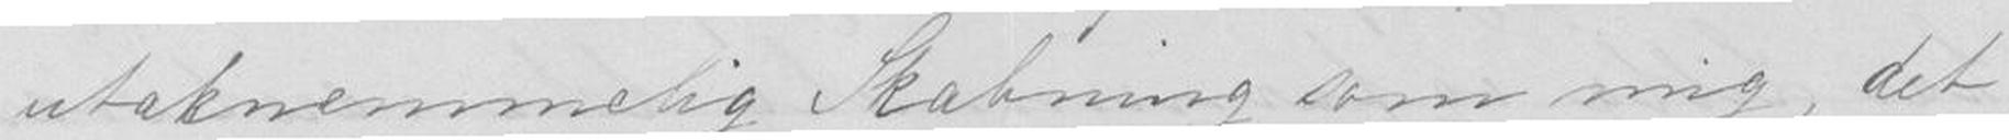utaknemmelig Skabning som mig, det
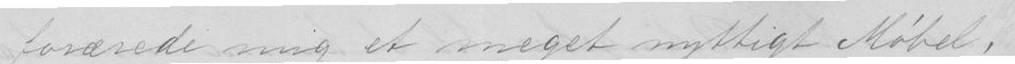forærede mig et meget nyttigt Møbel,
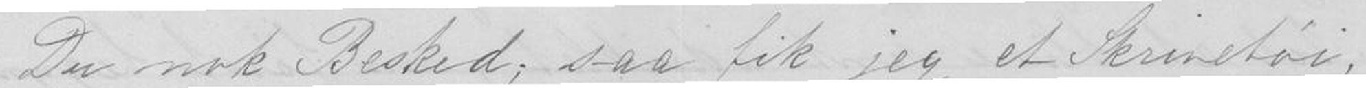Du nok Besked; saa fik jeg et skrivetøi.
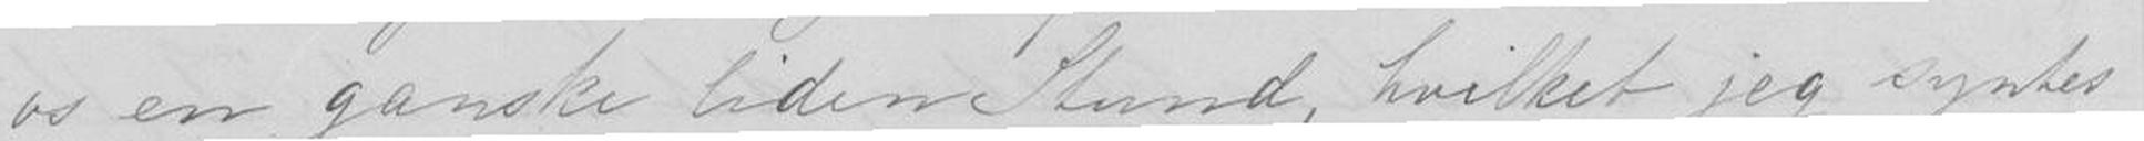os en ganske liden Stund, hvilket jeg syntes
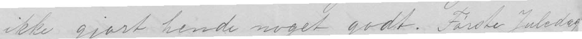ikke gjort hende noget godt. Først Juledag
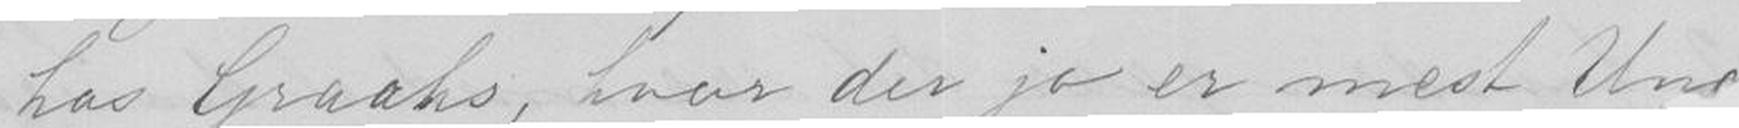hos Graahs, hvor der jo er mest un
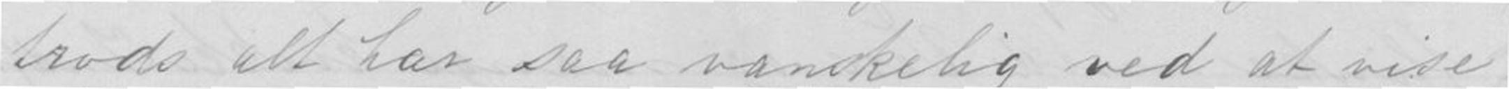brods alt har saa vanskelig ved at vise
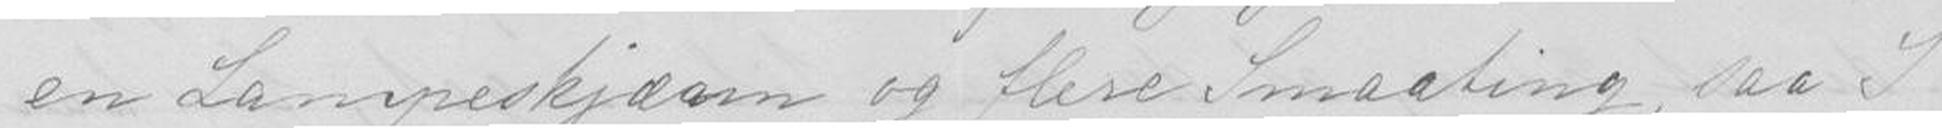en Lampeskjaarm og flere Smaating, saa I
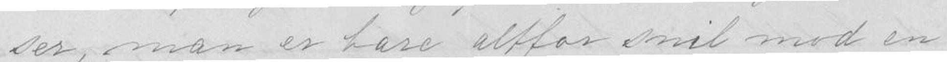ser, man er bare altfor snil mod en
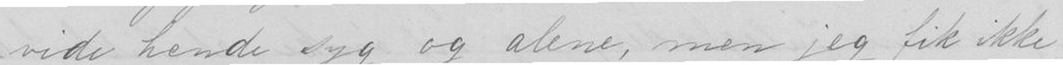vide hende syg og alene, men jeg fik ikke
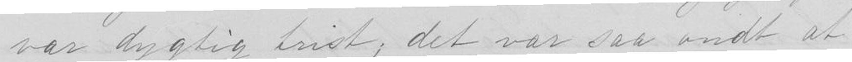var dygtig trist, det var saa ondt at
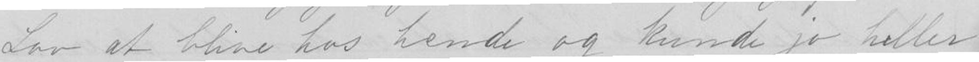Lov at blive hos hende og kunde jo heller
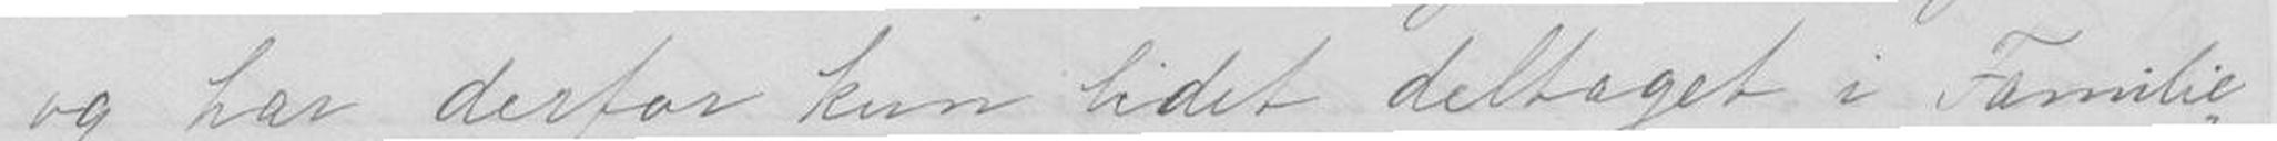og har derfor kun lidet deltaget i Familie-
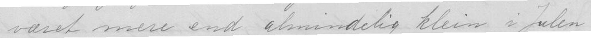været mere end almindelig klein i Julen
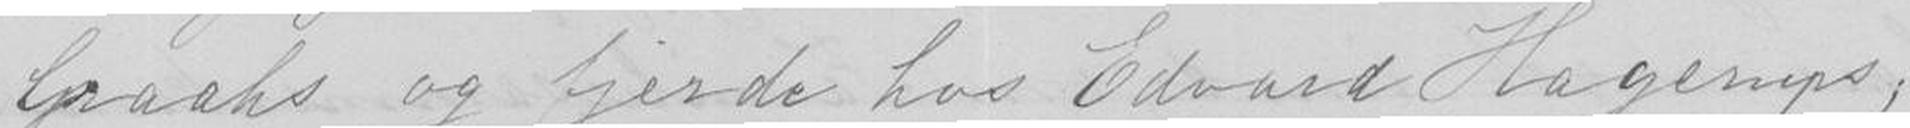Graahs og fjerde hos Edvardt Hagerneps,
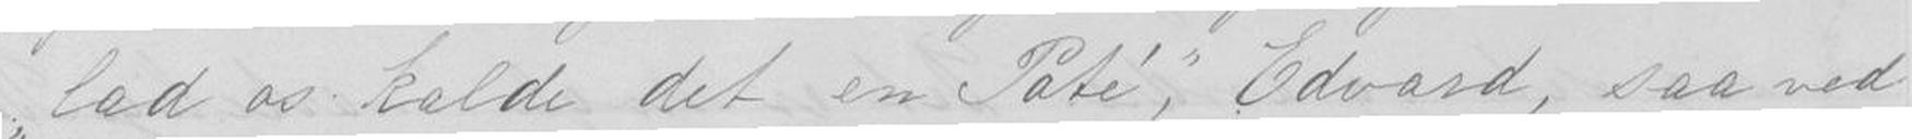bad os kalde det en Pate', Edvard, saa ved
